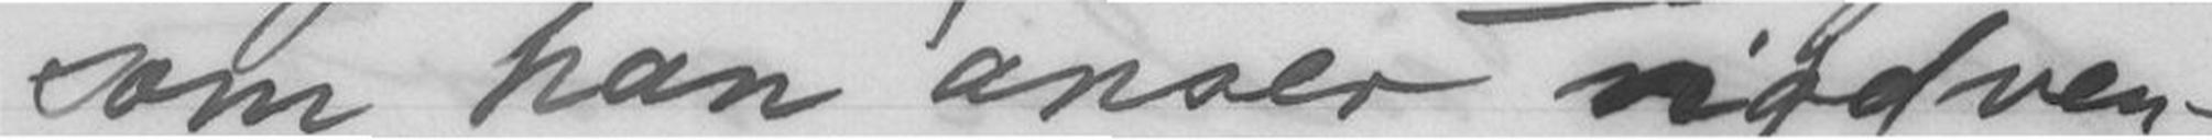som han anser nødven-
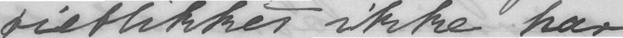øieblikket ikke har
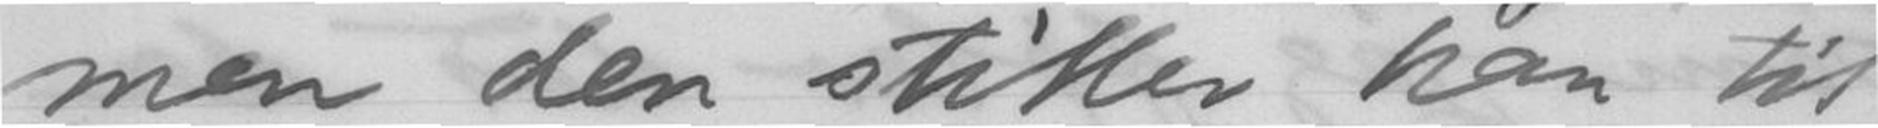men den stiller han til
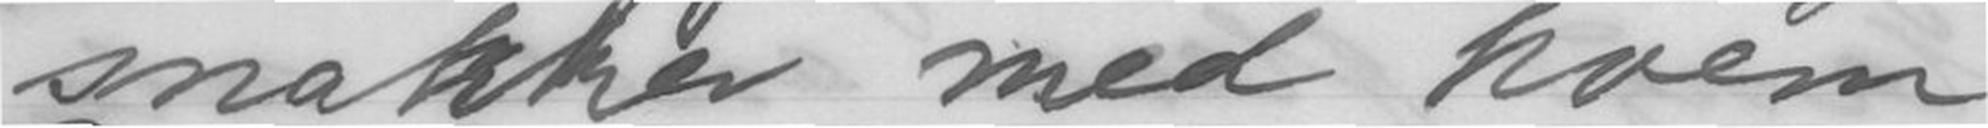snakker med hvem
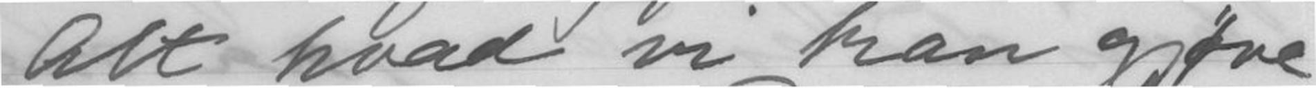Alt hvad vi kan gjøre
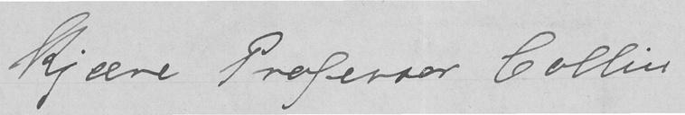Kjære Professor Collin
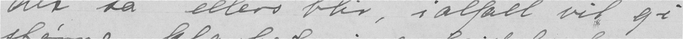det så ellers blir, ialfall vil gi
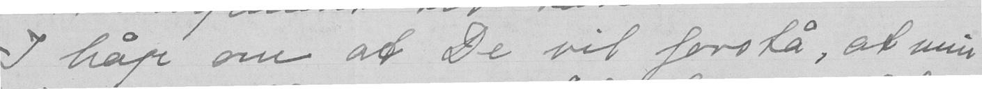I håp om at De vil forstå, at min
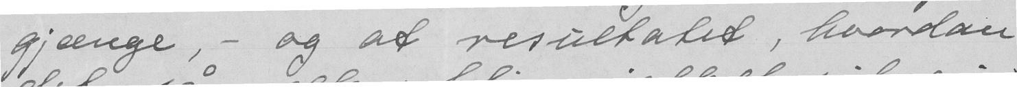gjænge, - og at resultatet, hvordan
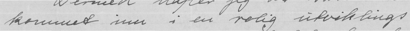kommet inn i en rolig udviklings-
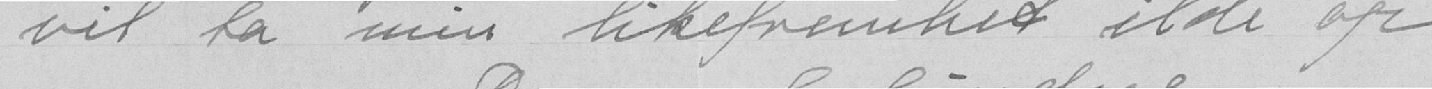vil ta min likefremhet ilde op
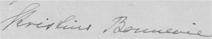Kristine Bonnevie
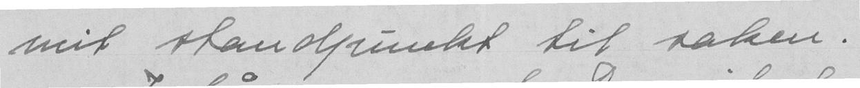mit standpunkt til saken.
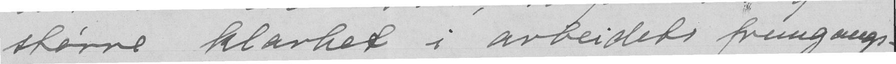større klarhet i arbeidets fremgangs-
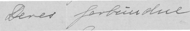Deres forbundne
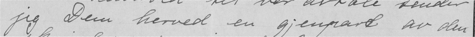jeg Dem herved en gjenpart av den
